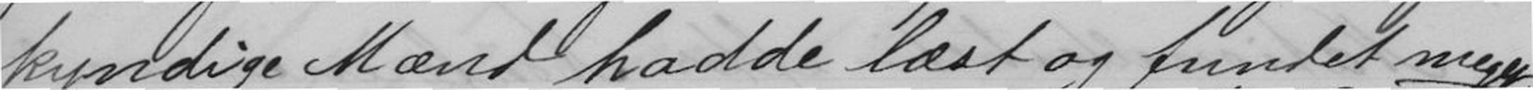kyndige Mænd hadde læst og fundet meget
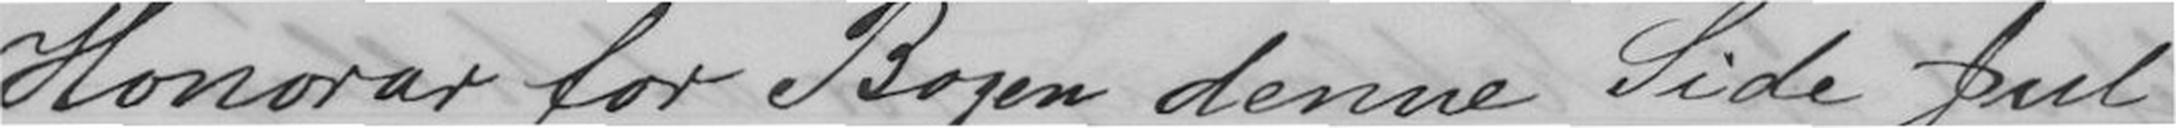Honorar for Bogen denne Side Jul
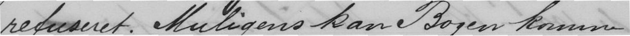refuseret. Muligens kan Bogen komme
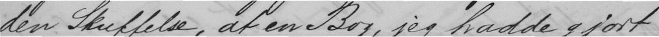den Skuffelse, at en Bog, jeg hadde gjort
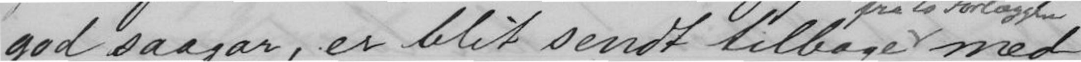god saagar, er blit sendt tilbage fra to Forlæggere med
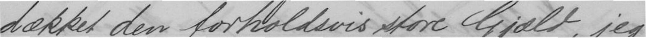dækket den forholdsvis store Gjæld, jeg
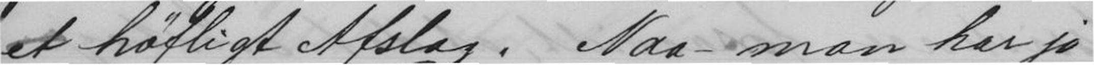et høfligt Afslag. Naa - man har jo
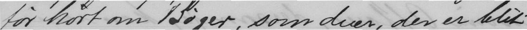før hørt om Bøger, som duer, der er blit
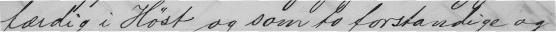færdig i Høst og som to forstandige og
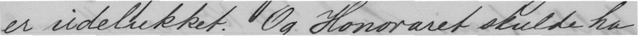er udelukket. Og Honoraret skulde ha
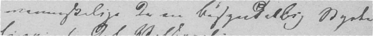menneskelige da en besynderlig Styrke
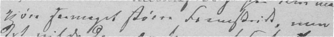gjøre saameget større Fremskridt, men
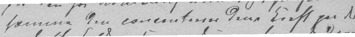hæmme den concentreres dens Kraft paa de
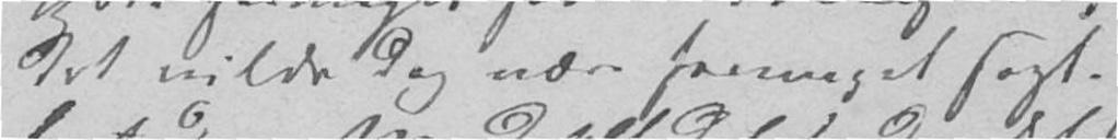det vilde dog være formeget sagt.
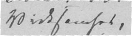Virksomhed,
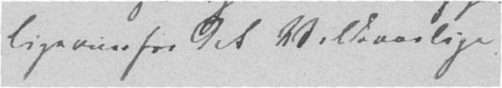ligeoverfor det Vilkaarlige.
